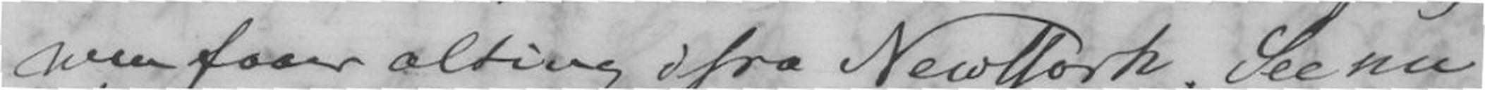men faaer alting ifra New York. Saa nu
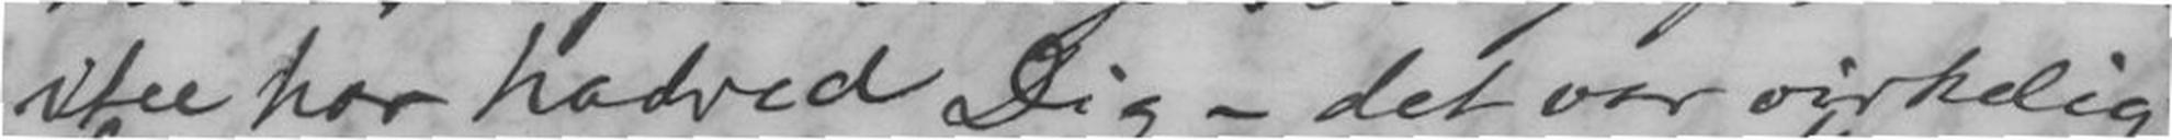itee har hadved Dig - det var virkelig
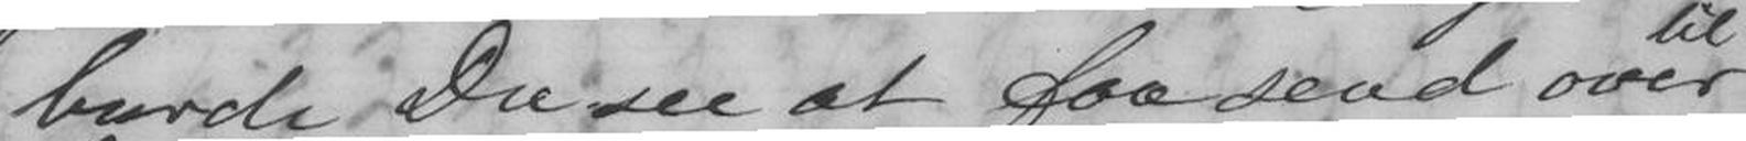burde Du see at faa send over
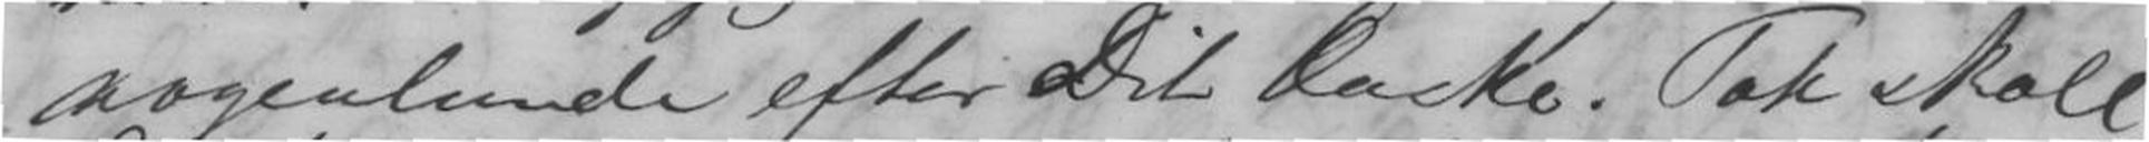nogenlunde efter Dit Ønske. Tak skall
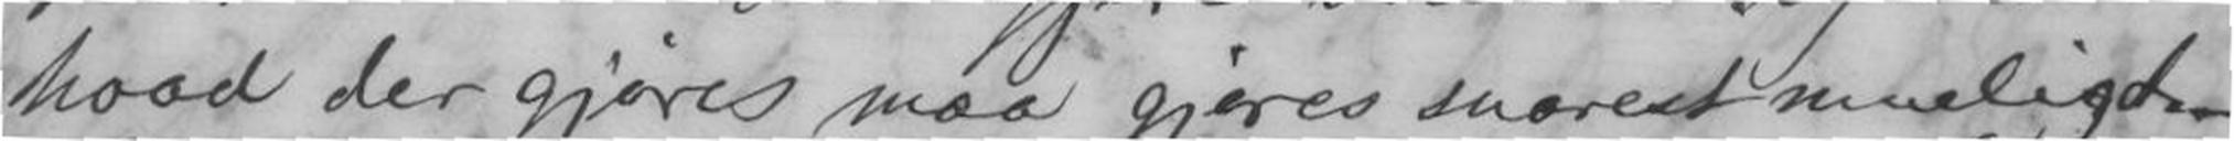hvad der gjæøres maa gjøres snarest mueligt.
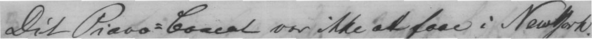Dit Piano-Concert var ikke at faae i New York;
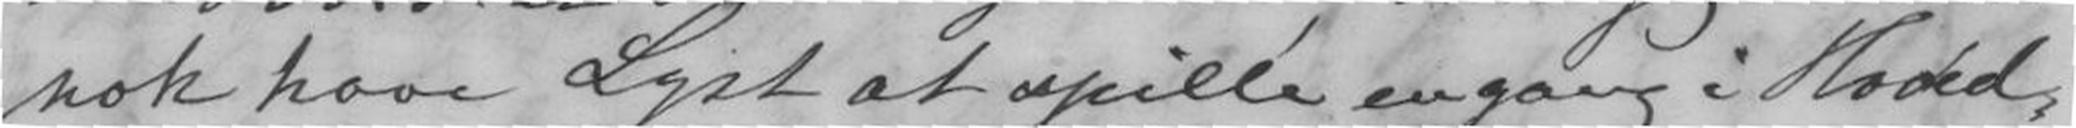nok have Lyst at spille engang i Hoved-
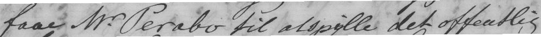faaer Mr Perabo til atspille det offentlig
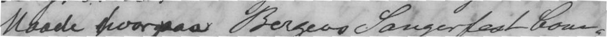Maade hvorpaa Bergens Sangerfest Com-
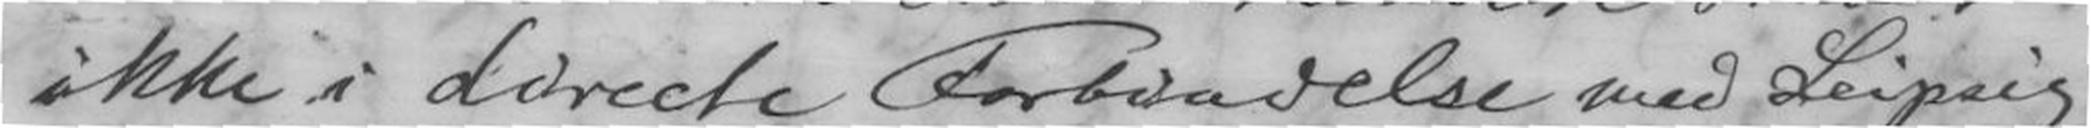ikke i directe Forbindelse med Leipzig
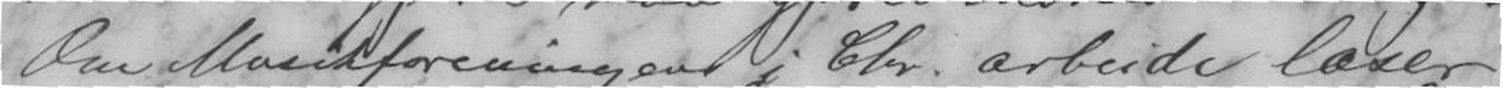Om Musikforeningen i Chr. arbeide læser
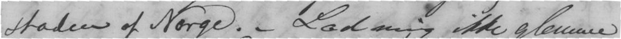staden af Norge. - Lad mig ikke glemme
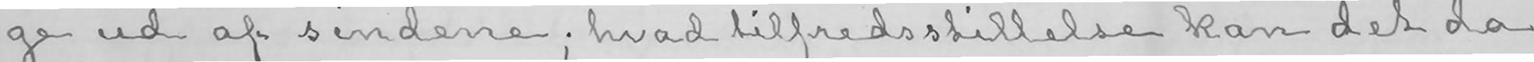ge ud af sindene; hvad tilfredsstillelse kan det da
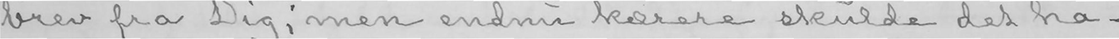brev fra Dig; men endnu kærere skulde det ha-
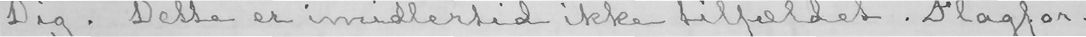Dig. Dette er imidlertid ikke tilfældet. Flagfor-
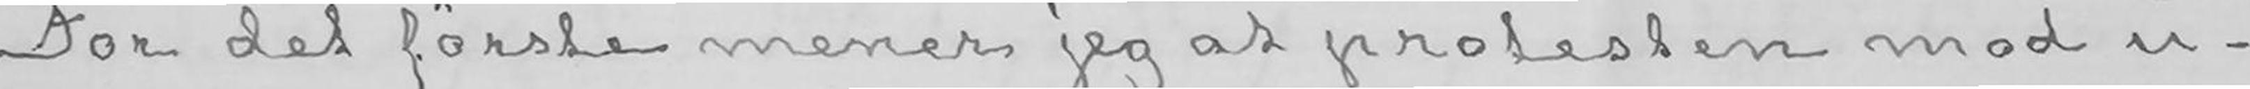For det første mener jeg at protesten mod u-
